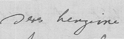Deres hengivne
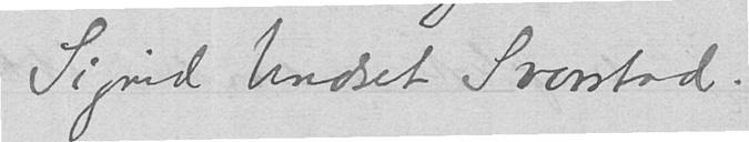Sigrid Undset Svarstad.
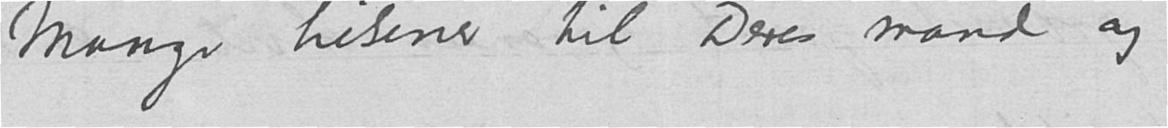Mange hilsener til Deres mand og
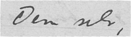Dem selv,
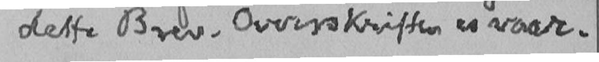dette Brev. Overrskriften er vaar.
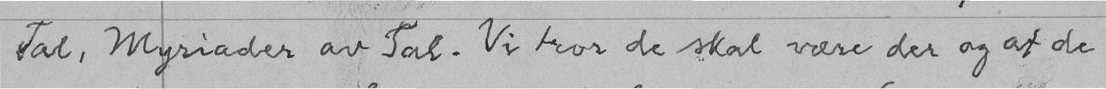Tal, Myriader av Tal. Vi tror de skal være der og at de
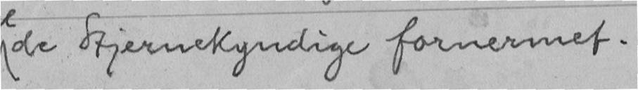de Stjernekyndige fornermet.
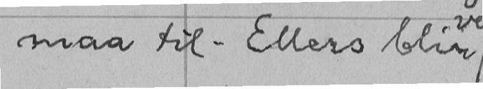maa til. Ellers blir
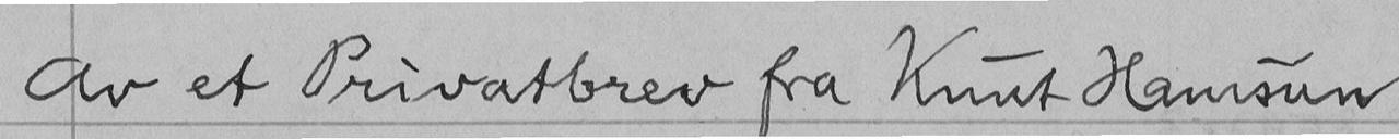Av et Privatbrev fra Knut Hamsun
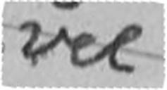vel
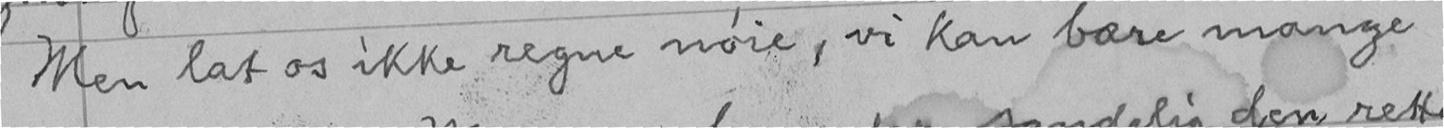Men lat os ikke regne nøie, vi kan bære mange
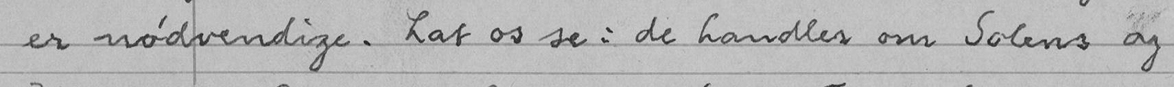er nødvendige. Lat os se: de handler om Solens og
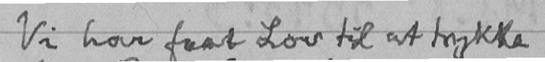Vi har faat Lov til at trykke
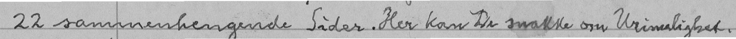22 sammenhengende Sider. Her kan De snakke om Urimelighet.
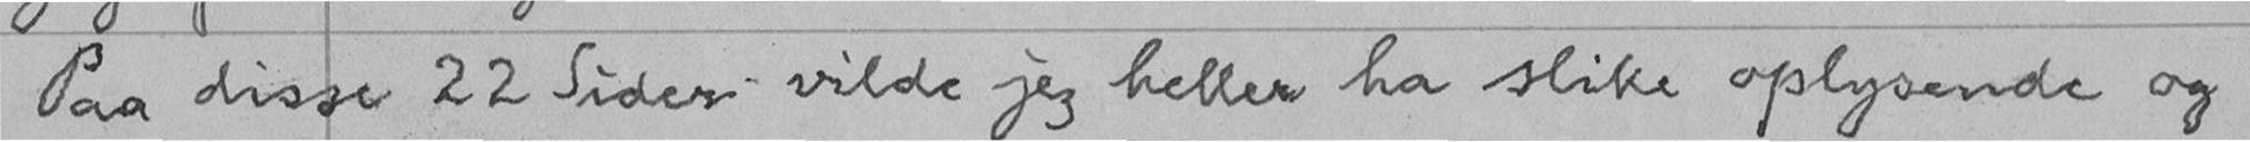Paa disse 22 Sider vilde jeg heller ha slike oplysende og
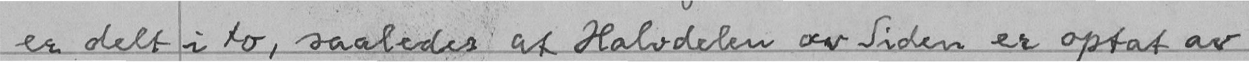er delt i to, saaledes at Halvdelen av Siden er optat av
